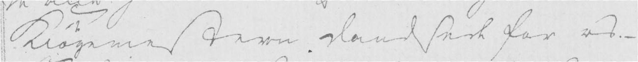Kiøgemesteren dandsede for os. -
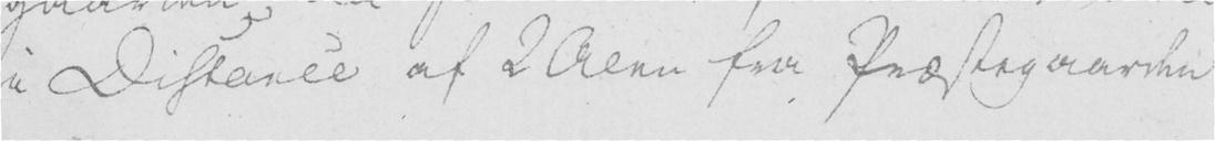i Distance af 2 Alen fra Præstegaarden
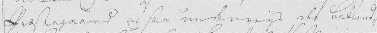Præstegaard og saa underveys det bekiend-
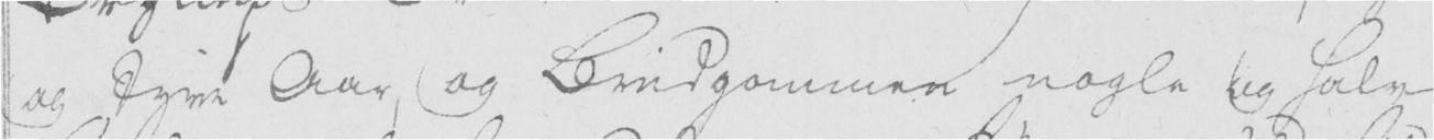og Tyve Aar, og Brudgommen nogle og halv-
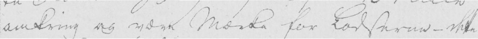omkring og være Mærke for Lodserne - dette
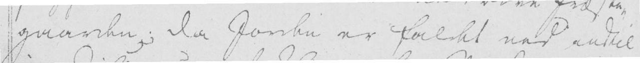gaarden; da Jorden er faldet ned indtil
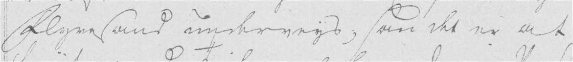Flyvesand underveys, som det er at
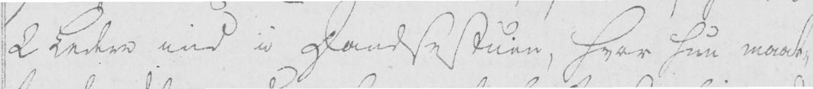2 Ledere ind i Dandsestuen, hvor hun maat-
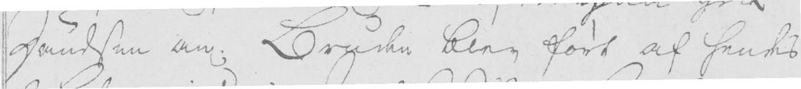Dandsen om; Bruden blev ført af hendes
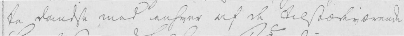te dandse med enhver af de tilstædeværende
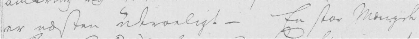er næsten utroeligt - En stor Mængde
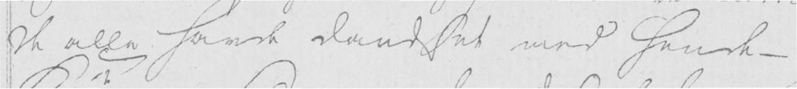de alle havde dandset med hende -
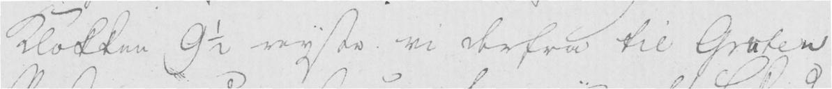Klokken 9 1/2 reyste vi derfra til Groten
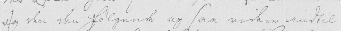og den den følgende og saa videre indtil
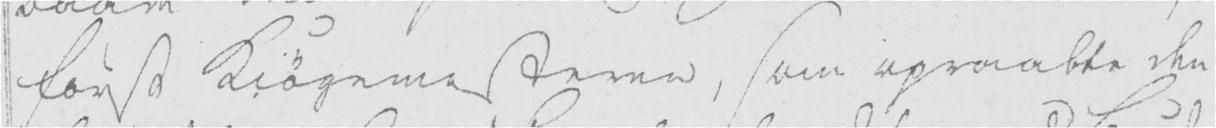først Kiøgemesteren, som opraabte den
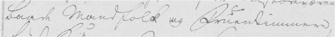baade Mandfolk og Fruentimmere,
